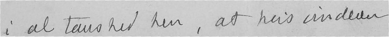i al taushed her, at hvis vinderen
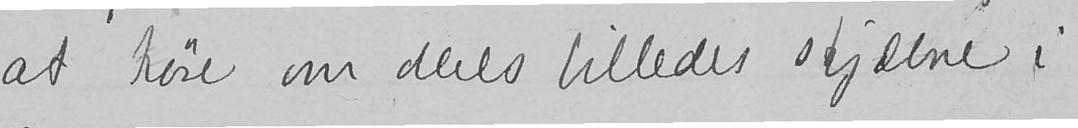at høre om deres billedes skjæbne i
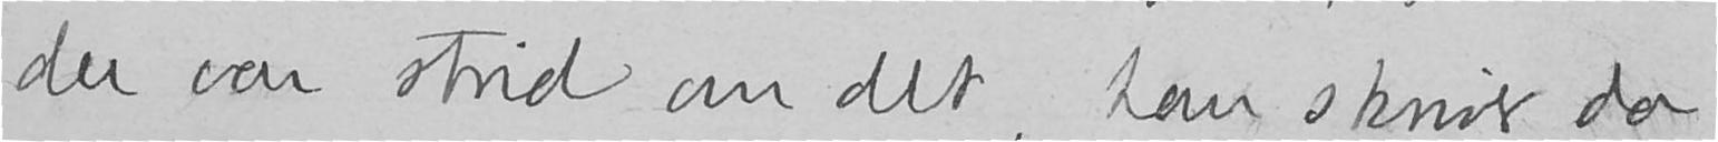der var strid om det, han skriver da
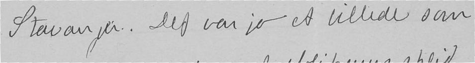Stavanger. Det var jo et billede som
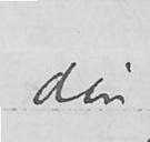din
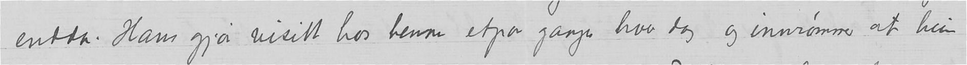endda. Hans gjør visitt hos henne etpar ganger hver dag og innrømmer at hun
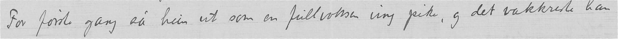For første gang så hun ut som en fullvoksen ung pike, og det vakkreste han
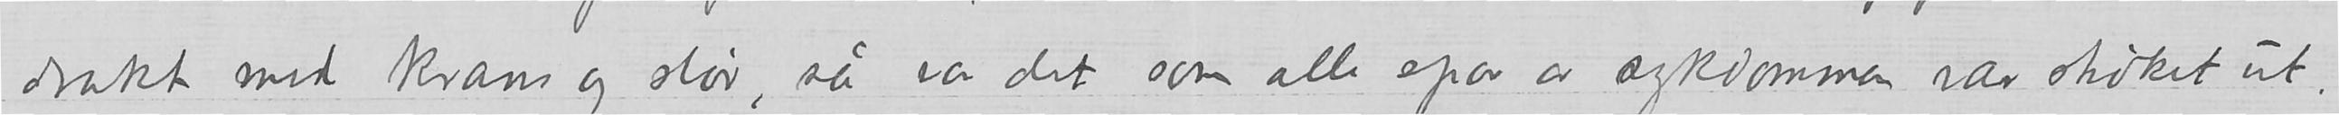drakt med krans og slør, su var det som alle spor av sykdommen var strøket ut,
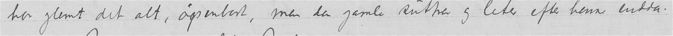har glemt det alt, åpenbart, men den gamle suttrer og leter efter henne endda.
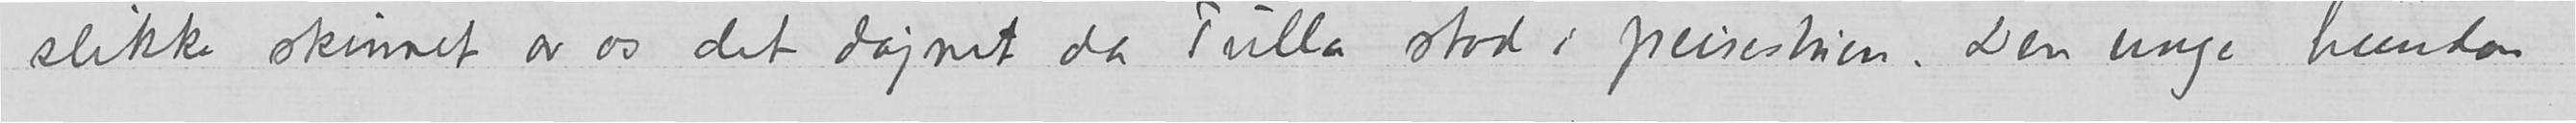slikke skinnet av os det døgnet da Tulla stod i peisestuen. Den unge hunden
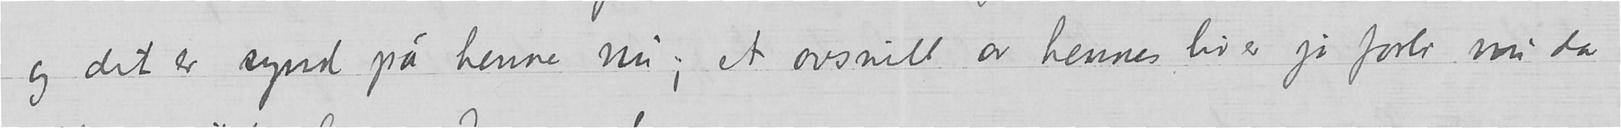og det er synd pa henne nu; et avsnitt av hennes liv er jo forbi nu da
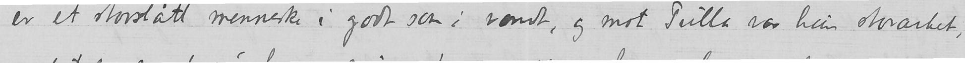er et storslått menneske i godt som i vondt, og mot Tulla var hun storartet,
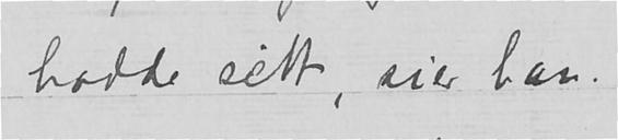hadde sitt, sier han.
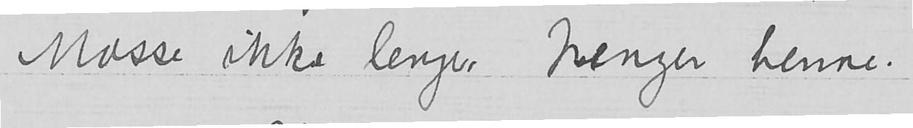Mosse ikke lenger trenger henne.
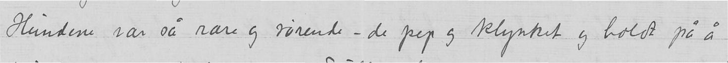Hundene var så rare og rørende – de pep og klynket og holdt på å
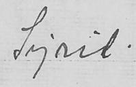Sigrid.
